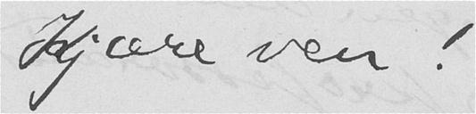Kjære ven!
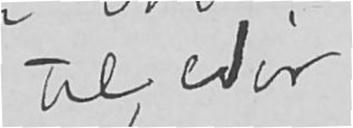til eder
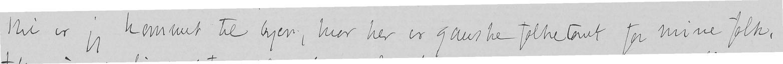Nu er jeg kommet til byen, hvor her er ganske folketomt for mine folk,
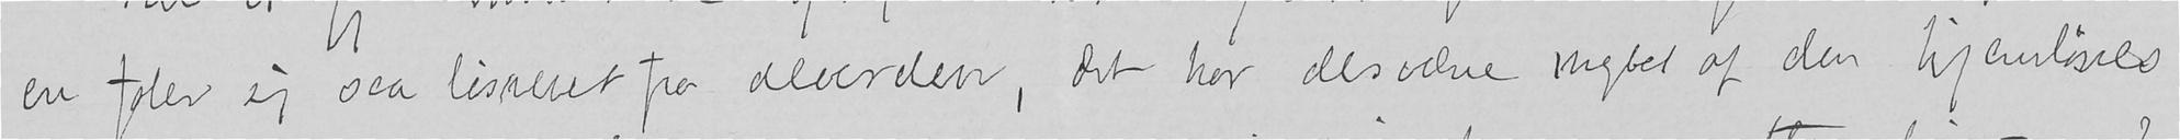en føler sig saa løsrevet fra alverden, det har desværre meget af den hjemløses
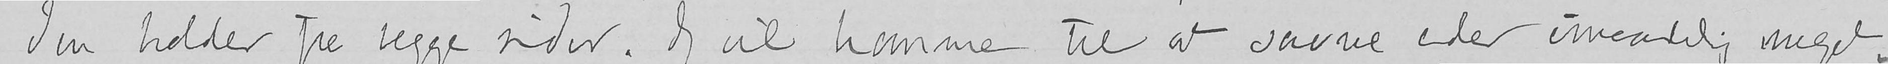den holder fra begge sider. Jeg vil komme til at savne eder umaadelig meget
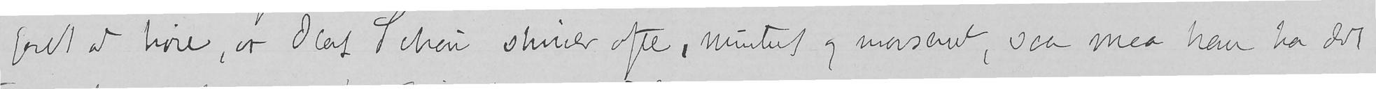Godt at høre, at Olaf Schou skriver ofte, muntert og morsomt, saa maa han ha det
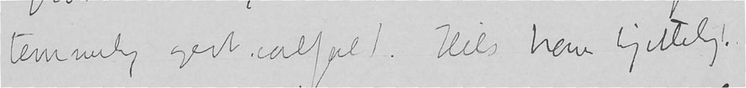temmelig godt ialfald. Hils han hjertelig.
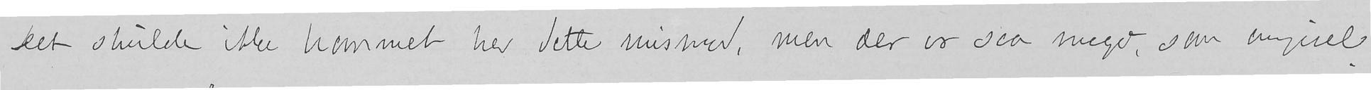Det skulde ikke kommet her dette mismod, men der er saa meget, som omgivel-
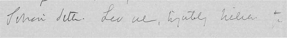Schou dette. Lev vel, hjertelig hilsen fra
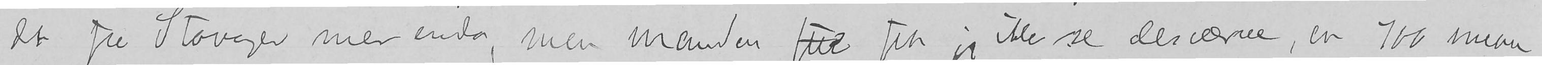det fra Stavanger mer enda, men manden fiik fik jeg ikke se desværre, en 100 menne-
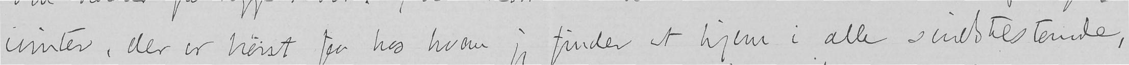ivinter, der er høist faa hos hvem jeg finder et hjem i alle sindstilstande,
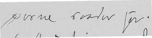serne raader for.
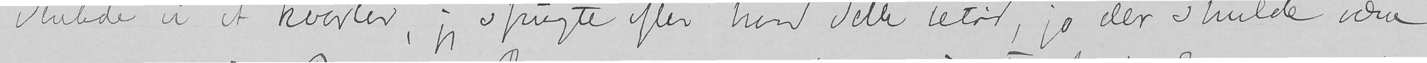ventede vi et kvarter, jeg spurgte efter hvad dette betød, jo der skulde være
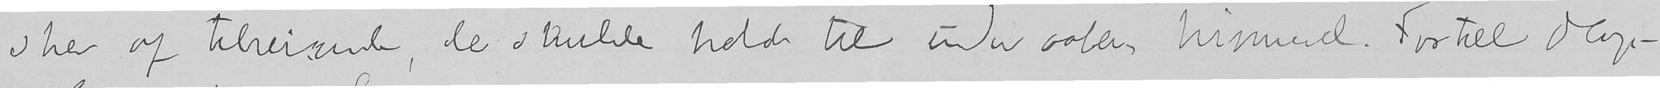sker af tilreisende, de skulde holde til under aaben himmel. Fortæl Oluf
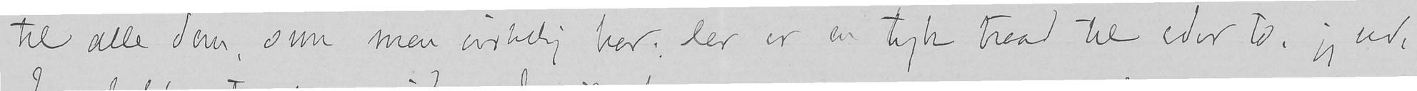til alle dem, som man virkelig har, der er en tyk traad til eder to, jeg ved,
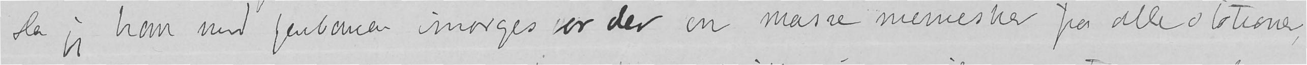Da jeg kom med jernbanen imorges var der en masse mennesker fra alle stationer,
